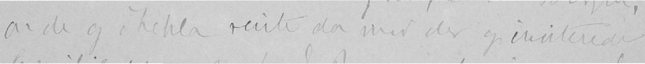oncle og Thekla reiste da med der og inviterede
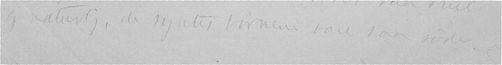og naturlig, de syntes børnene bare saa søde.
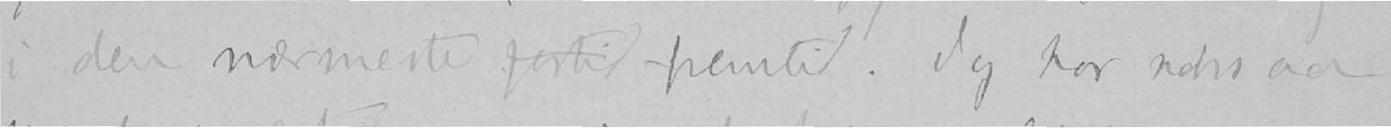i den nærmeste fortid fremtid. Jeg har noksaa
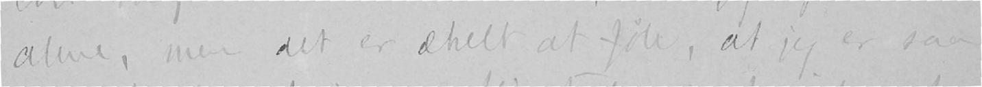alene, men det er ækelt at føle, at jeg er saa
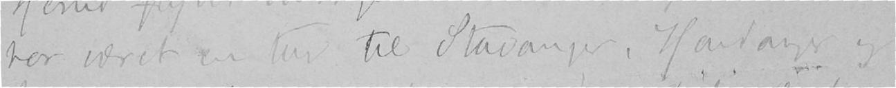har været en tur til Stavanger, Hardanger og
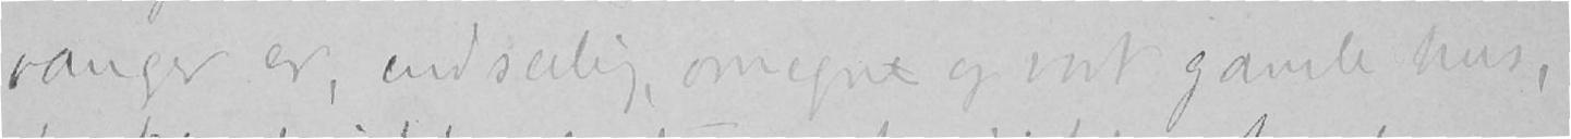vanger er, indseiling, omegne og vort gamle hus,
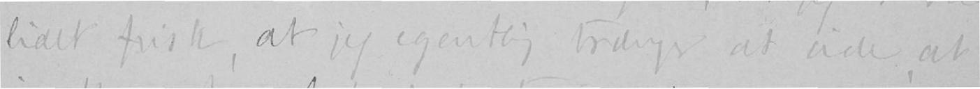lidet frisk, at jeg egentlig trænger at vide, at
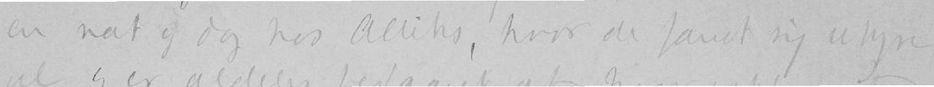en nat og dag hos Alliks, hvor de fandt sig uhyre
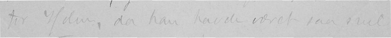for Holm, da han havde været saa snil
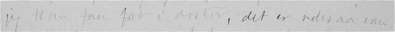jeg kan faa fat i doctor, det er noksaa van
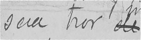saa tror de
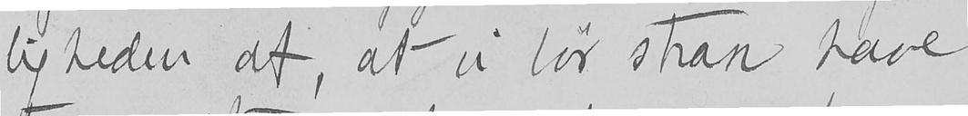ligheden af, at vi bør strax have
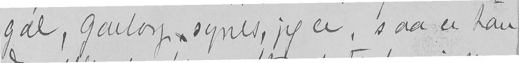gal, Garborg synes, jeg er, saa er han
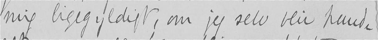mig ligegyldigt, om jeg selv blir hund-
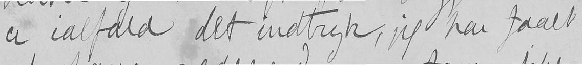er ialfald det indtryk, jeg har faaet
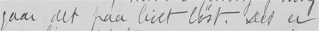gaar det paa livet løst. Det er
